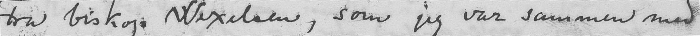Fra biskop Wexelsen, som jeg var sammen med
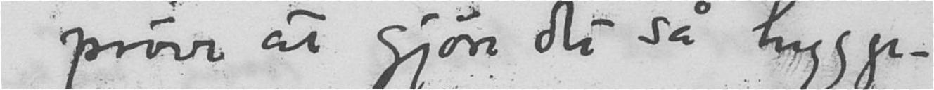prøve at gjøre det så hygge-
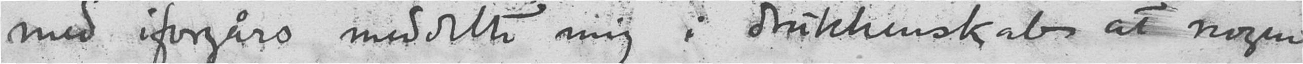med iforgårs meddelte mig i drukkenskab at nogen
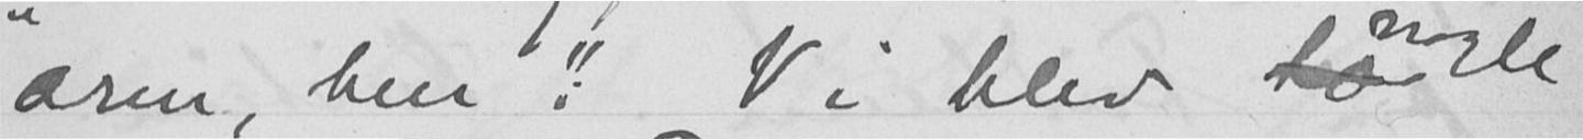arm, ben". Vi blev to nogle
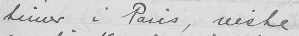timer i Paris, reiste
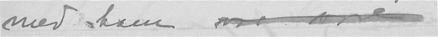med ham var vore
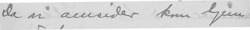Da vi omsider kom hjem
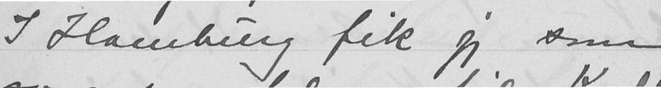I Hamburg fik jeg som
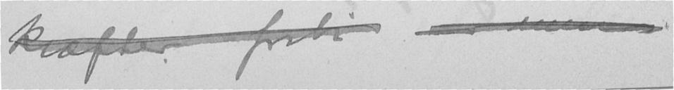kræfter forbi men
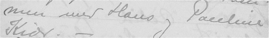men med Hans og Pauline
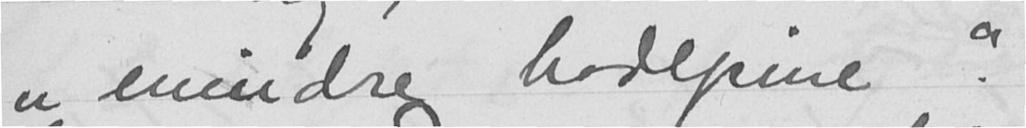"mindre hodepine".
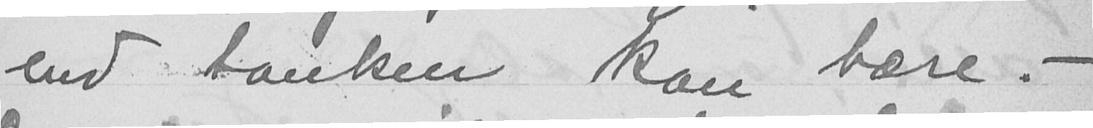end tanken kan bære. -
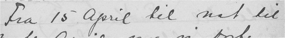Fra 15 April til nat til
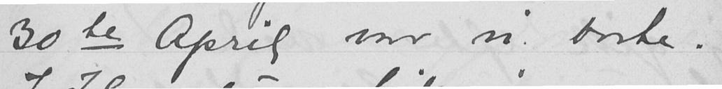30te April var vi borte.
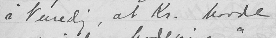i Venedig, at Kr. havde
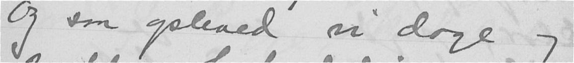Og saa opleved vi dage og
